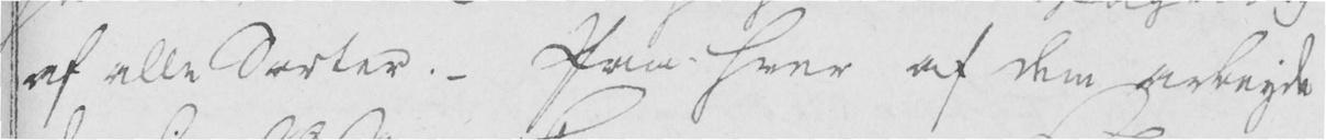af alle Sorter. - Paa hver af dem arbeyde
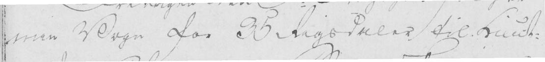min Vogn for 35 Rigsdaler til Lieut-
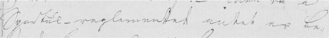Sportul-reglementet intet er be-
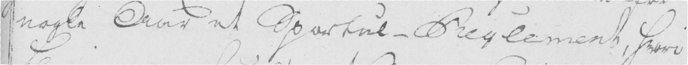nogle Aar et Sportul-Reglement, hvori
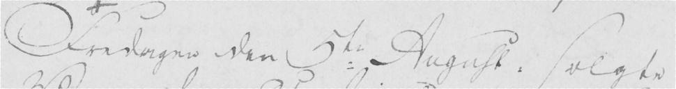Fredagen den 5te August: solgte
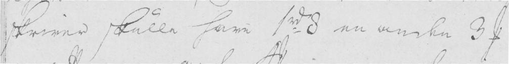skriver skulle have 1 rde 8 en anden 3 s
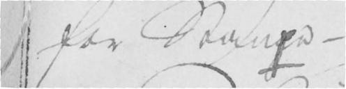for Saaepe -
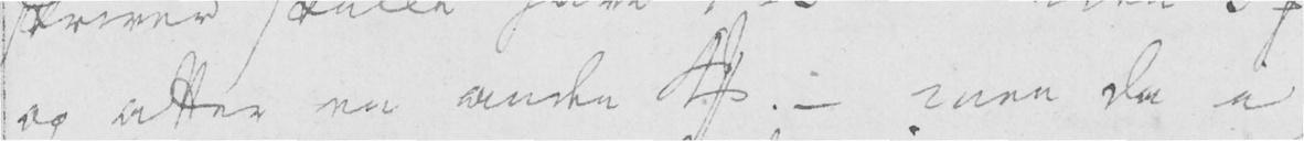og atter en anden 4 s. - men da i
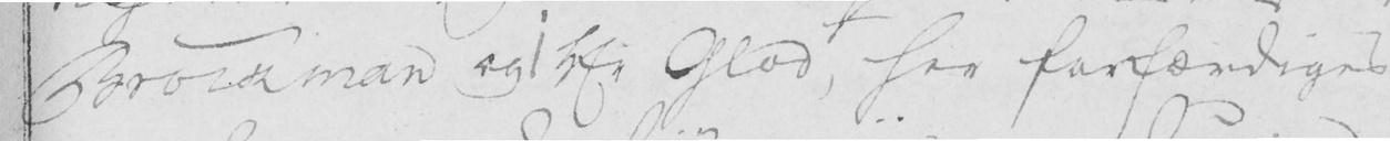Brockman og Hr Glad, her forfærdiges
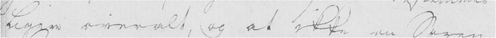ligen overalt, og at ikke en Soren-
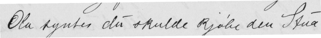Ola syntes du skulde kjøbe den Stua
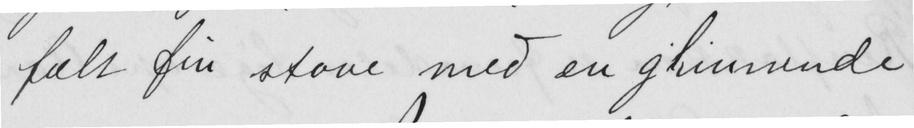fælt fin stove med en glimrende
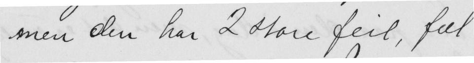men den har 2 store feil, ful
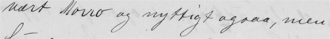vært Morro og nyttigt ogsaa, men
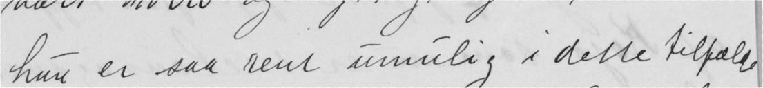hun er saa rent umulig i dette tilfælde
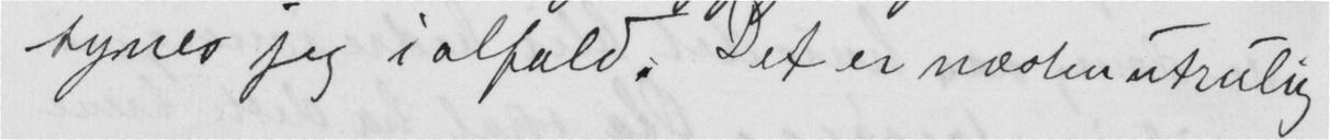synes jeg ialfald. Det er næsten utrulig
